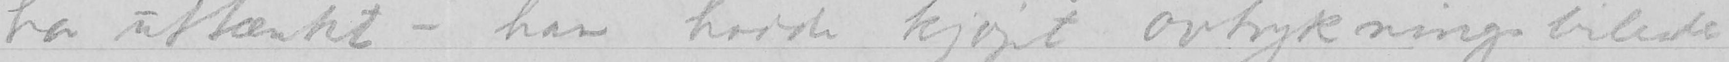har uttænkt – han hadde kjøpt <avtrykningsbileder>
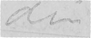din
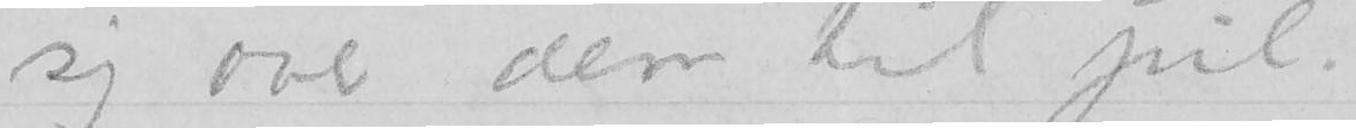sig over dem til jul.
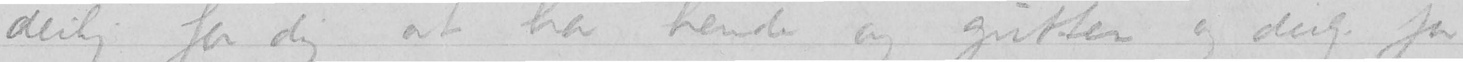deilig for dig at ha hende og gutten og deilig for
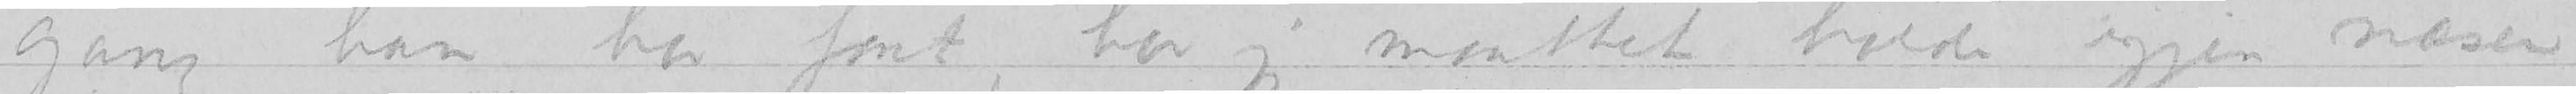gang han har faat, har jeg maattet holde igjen næsen
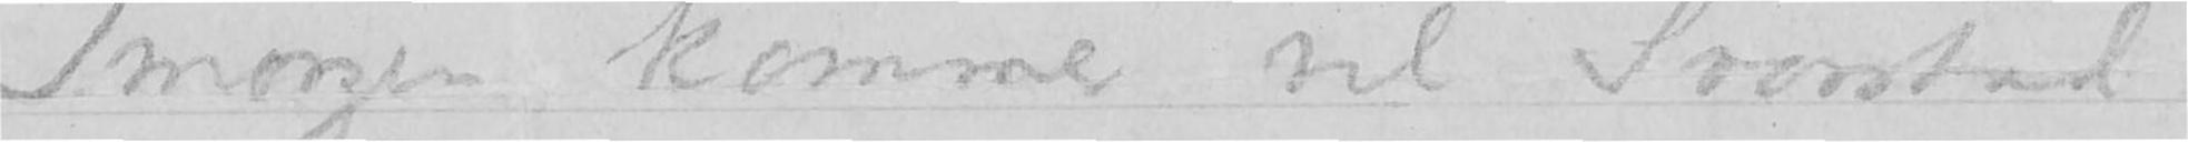Imorgen kommer vel Svarstad –.
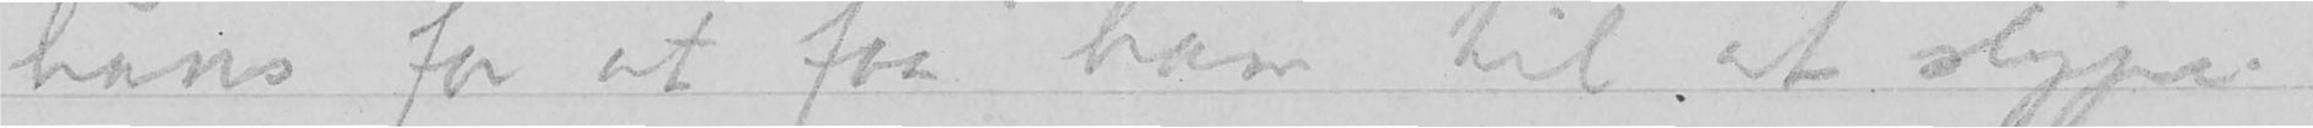hans for at faa ham til at slippe.
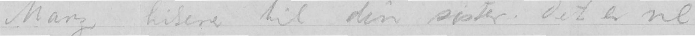Mange hilsener til din søster. Det er vel
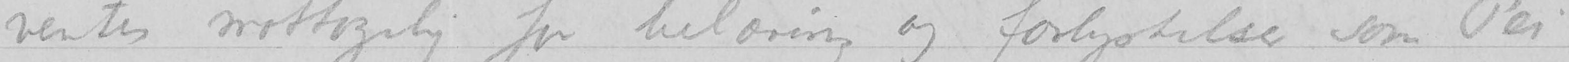ventes mottagelig for belæring og forlystelser som Pei
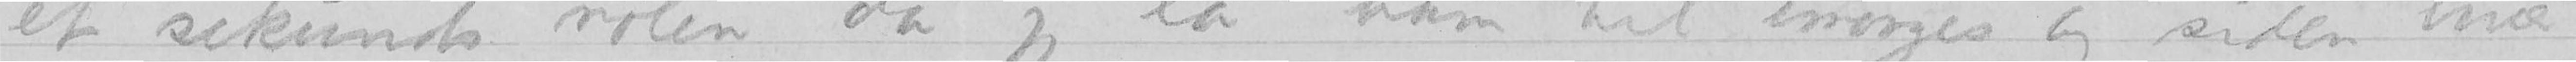et sekunds nølen, da jeg la ham til imorges og siden hvær
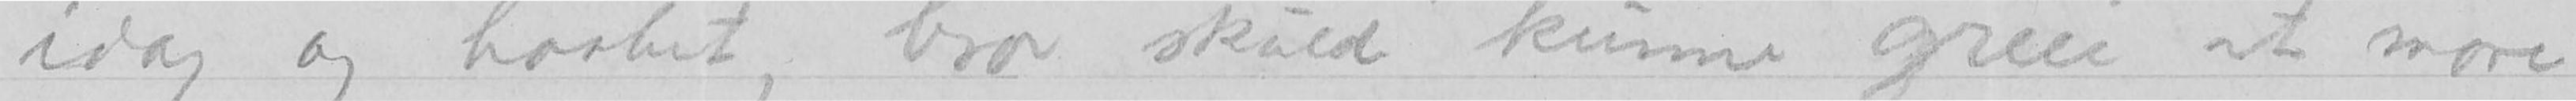idag og haabet, bror skulde kunne greie at more
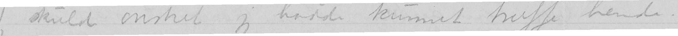Jeg skulde ønsket jeg hadde kunnet træffe hende.
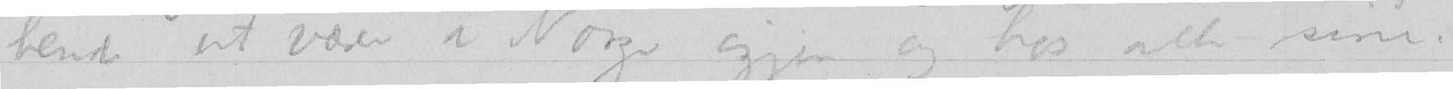hende at være i Norge igjen og hos alle sine.
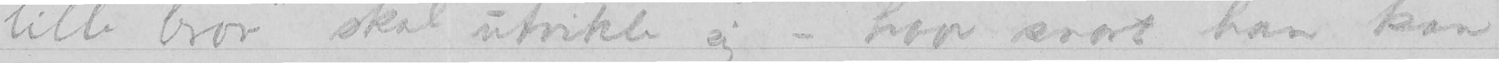lille bror» skal utvikle sig – hvor snart han kan
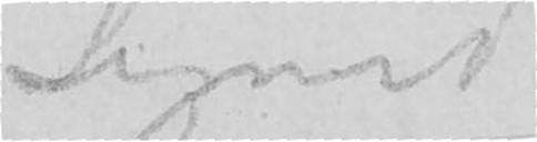Sigrid
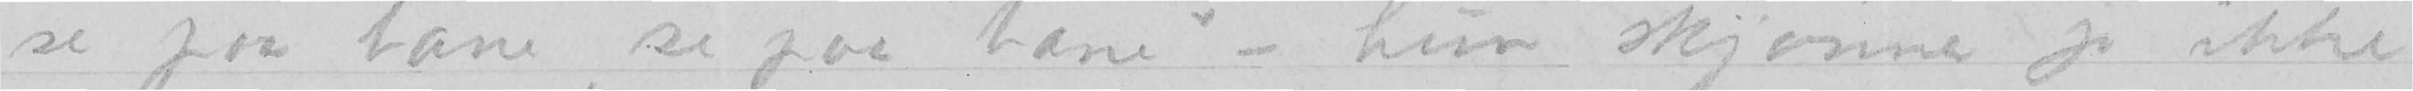«se paa ham, se paa ham» - hun skjønner jo ikke
